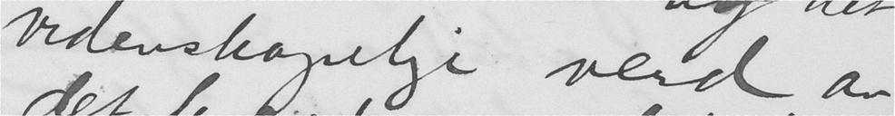videnskapelige verd an-
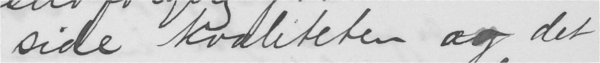side kvaliteten og det
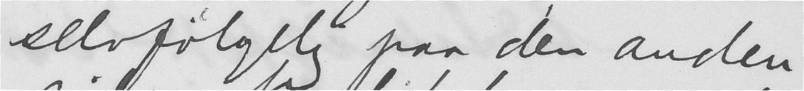selvfølgelig paa den anden
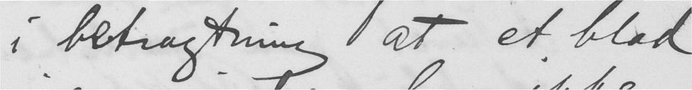i betragtning at et blad
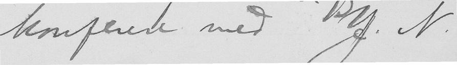konferere med Y. N.
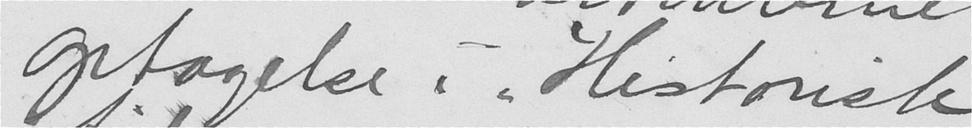optagelse i "Historisk
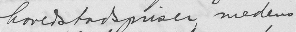hovedstadspriser, medens
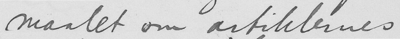maalet om artiklernes
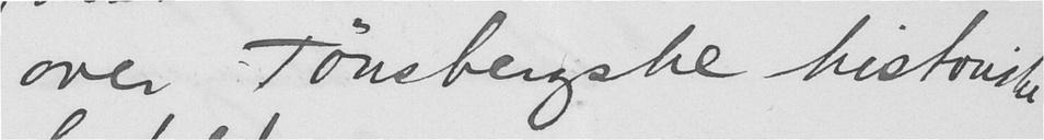over Tønsbergske historiske
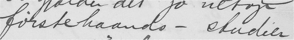førstehaands-studier
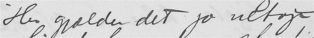Her gjælder det jo netop
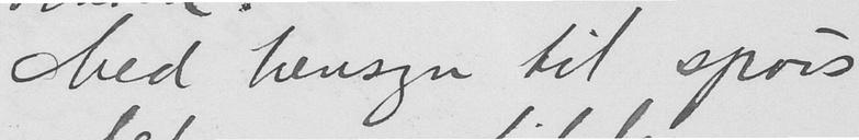Med hensyn til spørs-
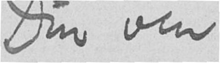Din ven
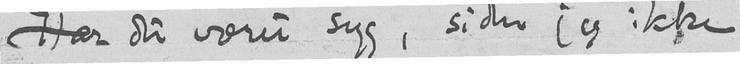Har du været syg, siden jeg ikke
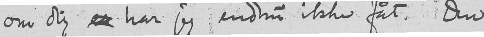om dig er har jeg endnu ikke fåt. Den
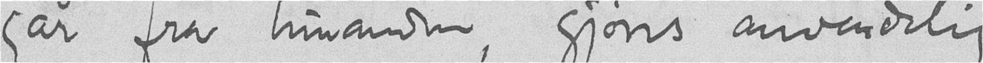går fra hinanden, gjøres anvendelig
